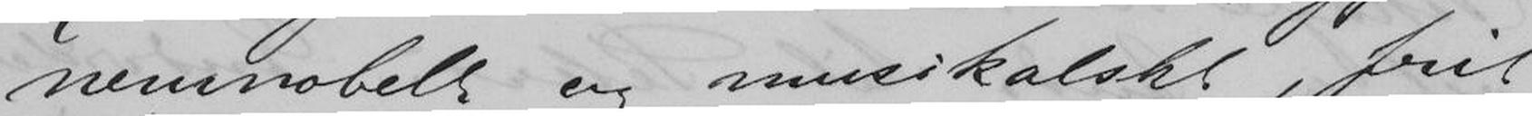nemnobelt og musikalskt, frit
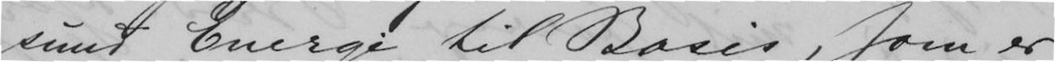sund Energi til Basis, som er
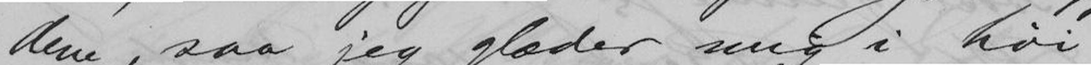dene,saa jeg glæder mig i høi
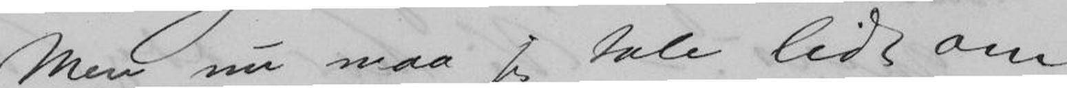Men nu maa jeg tale lidt om
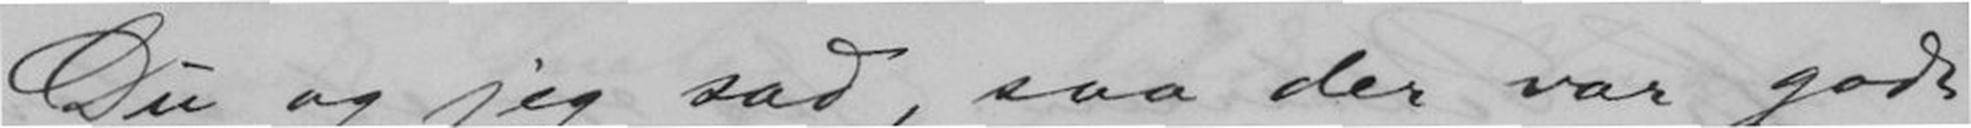Du og jeg sad, saa der var godt
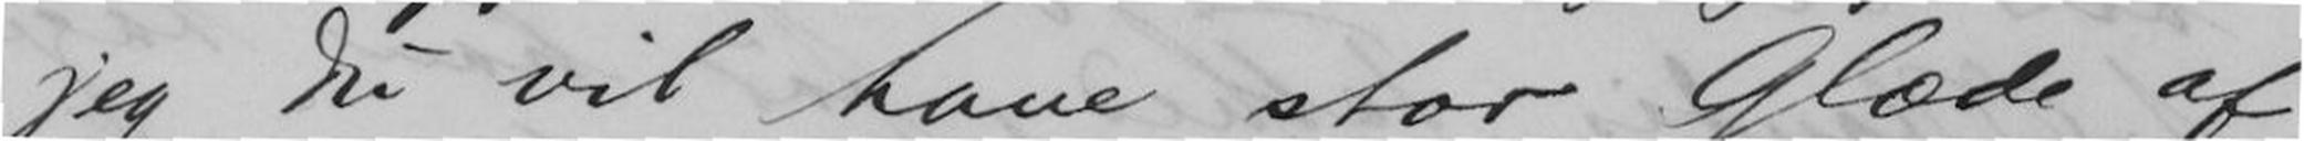jeg du vil have stor Glæde af
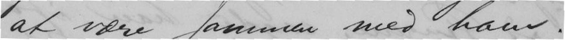at være sammen med ham. -
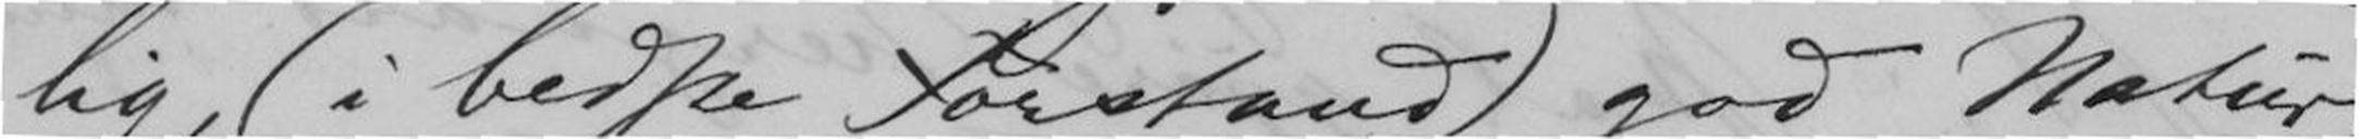lig, (i bedste Forstand) god Natur,
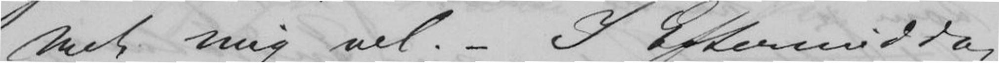met mig vel. - I Eftermiddag
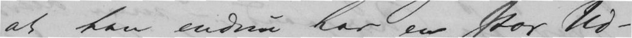at han endnu har en stor Ud-
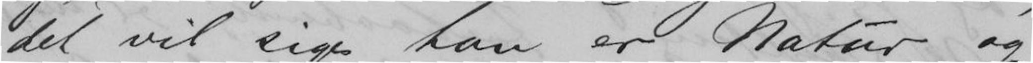det vil sige han er Natur og
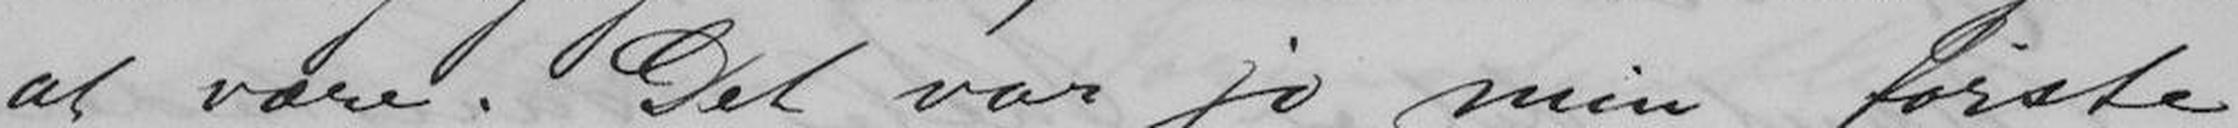at være. Det var jo min første
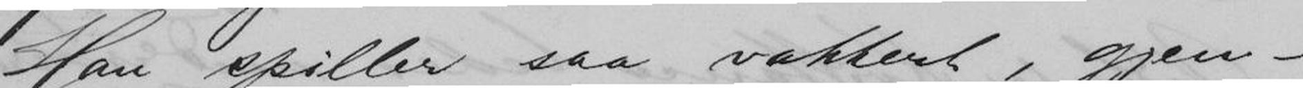Han spiller saa vakkert, gjen-
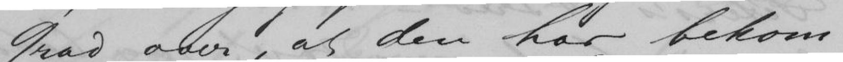Grad over, at den har bekom-
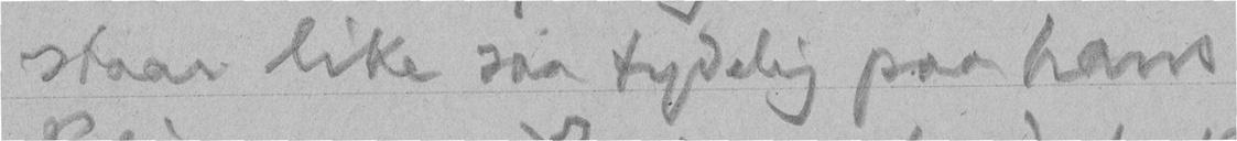staar like saa tydelig paa hans
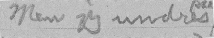Men jeg undres
